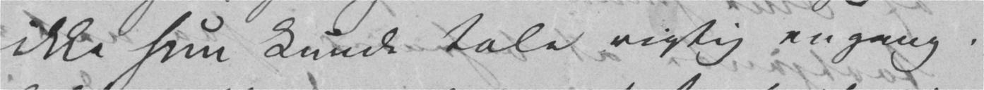ikke hun kunde tale rigtig engang.
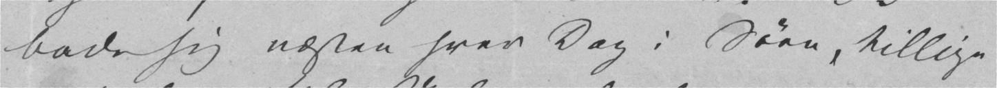bade sig næsten hver Dag i Søen, tillige
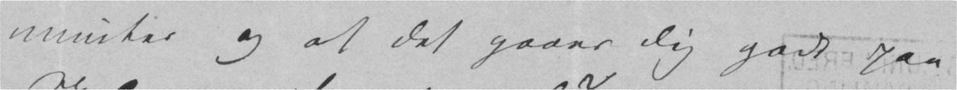munter og at det gaaer Dig godt paa
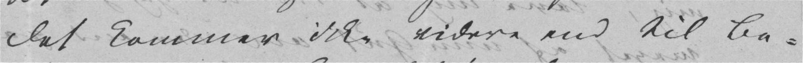det kommer ikke videre end til Be-
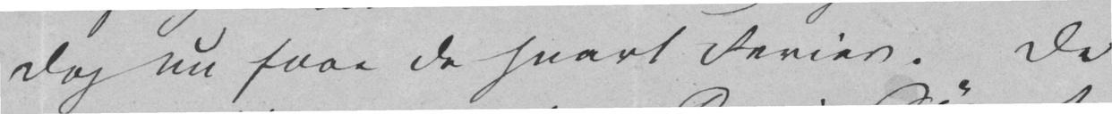dog nu faae de snart Ferier. De
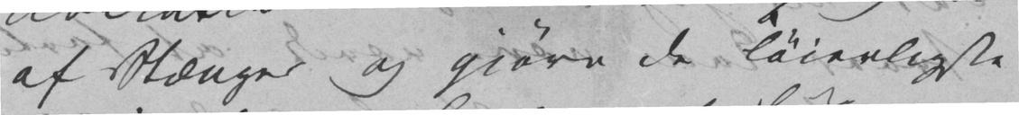af Stænger og gjøre de løierligste
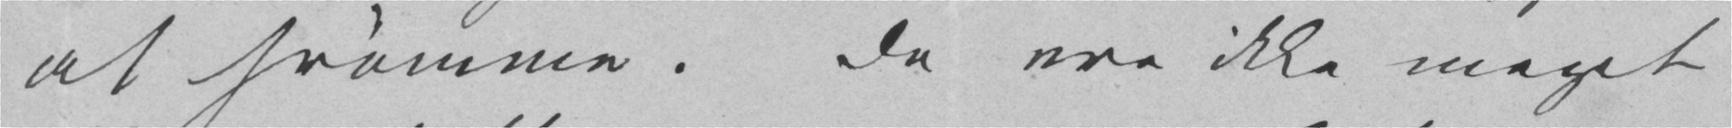at svømme. De ere ikke meget
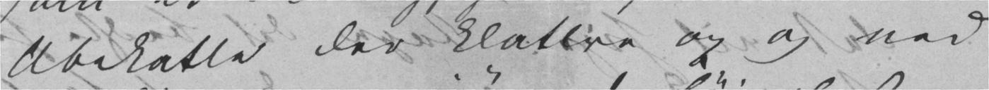Abekatte der klattre op og ned
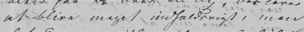at blive meget indholdsrigt, men
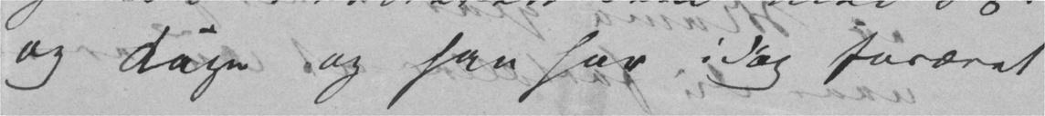og Kage og han har idag foræret
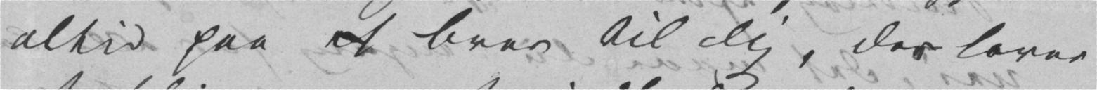altid paa et Brev til Dig, der lover
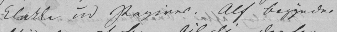klakke ud Papirer. Alf begynder
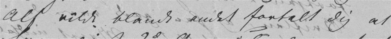Alf vilde blandt andet fortalt Dig at
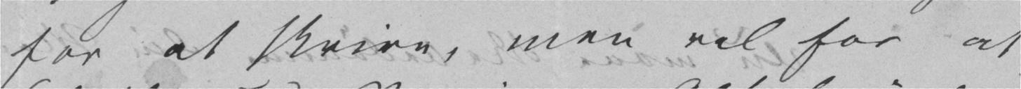for at skrive, men vel for at
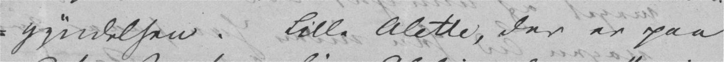gyndelsen. Lille Alette, der er paa
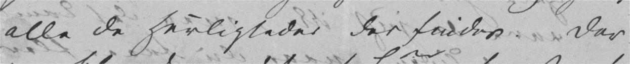alle de Herligheder der findes. Der
